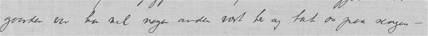gaarden vor har vel nogen anden vært her og tat os paa sengen –
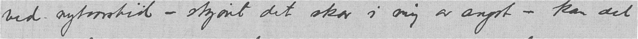ved nytaarstid - skjønt det skar i mig av angst – kan det
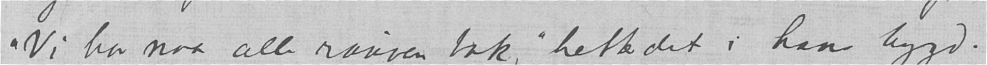"Vi har naa alle rauven bak," hetter det i hans bygd.
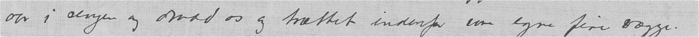aar i sengen og drad os og trættet indenfor vore egne fire vægge.
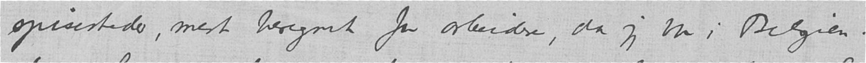spisesteder, mest beregnet for arbeidere, da jeg var i Belgien.
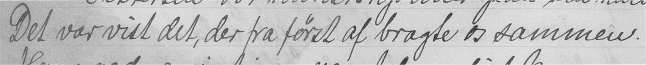Det var vist det, der fra først af bragte os sammen.
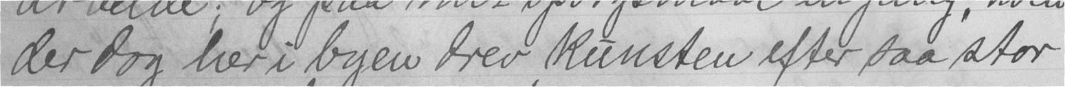der dog her i byen drev kunsten efter saa stor
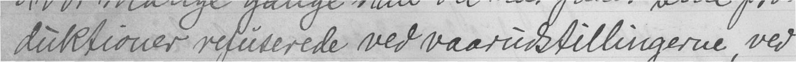duktioner refuserede ved vaarudstillingerne, ved
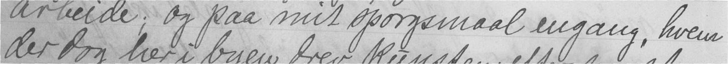arbeide; og paa mit spørgsmaal engang, hvem
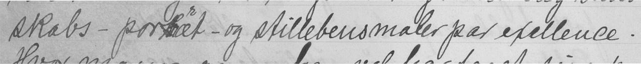skabs-portræt - og stillebensmaler par exellence.
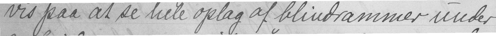vis paa at se hele oplag af blindrammer under
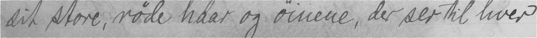sit store, røde haar og øiene, der ser til hver
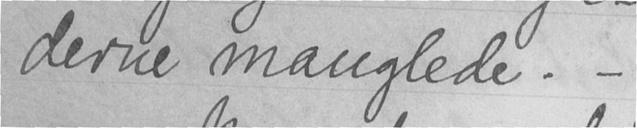derne manglede. -
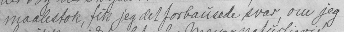maalestok, fik jeg det forbausede svar, om jeg
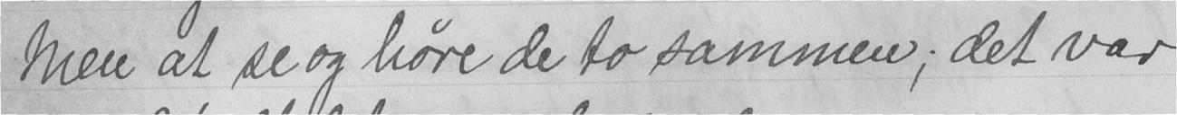Men at se og høre de to sammen; det var
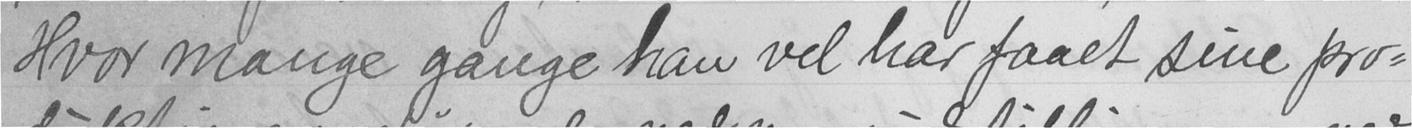Hvor mange gange han vel har faaet sine pro-
